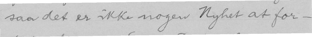saa det er ikke nogen Nyhet at for-
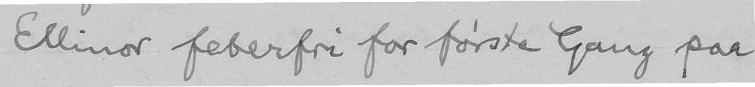Ellinor feberfri for første Gang paa
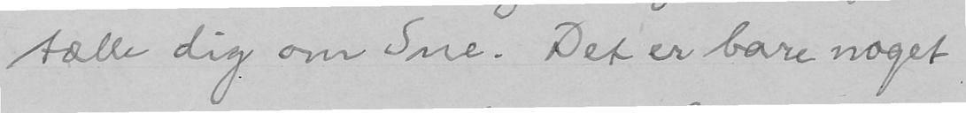tælle dig om Sne. Det er bare noget
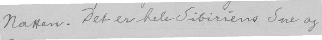Natten. Det er hele Sibiriens Sne og
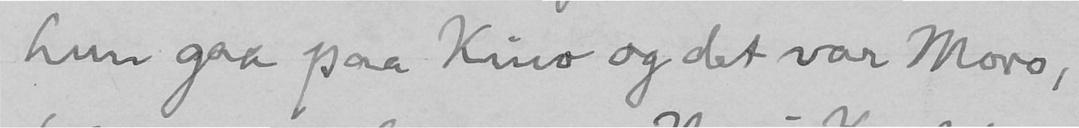hun gaa paa Kino og det var Moro,
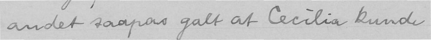andet saapas galt at Cecilia kunde
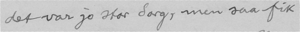det var jo stor Sorg, men saa fik
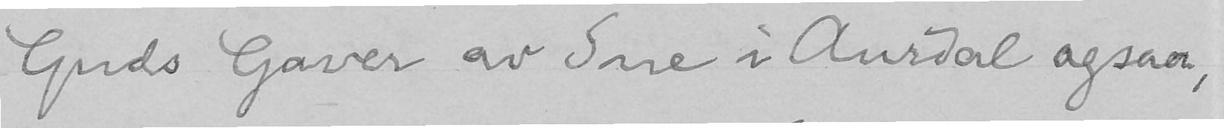Guds Gaver av Sne i Aurdal ogsaa,
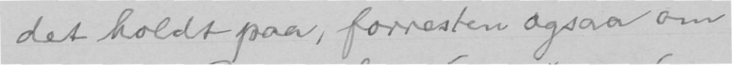det holdt paa, forresten ogsaa om
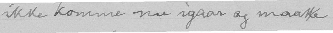ikke komme nu igaar og maatte
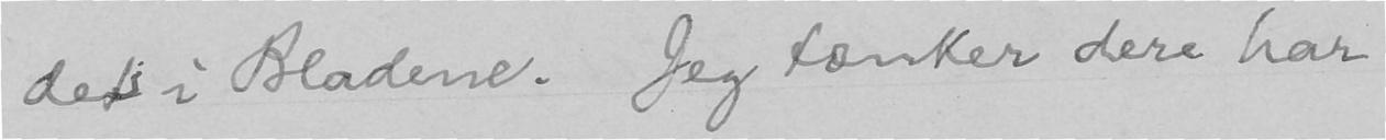det i Bladene. Jeg tænker dere har
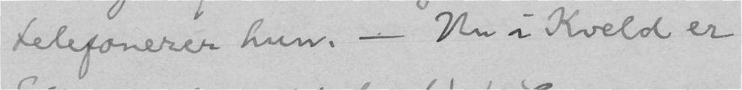telefonerer hun. - Nu i Kveld er
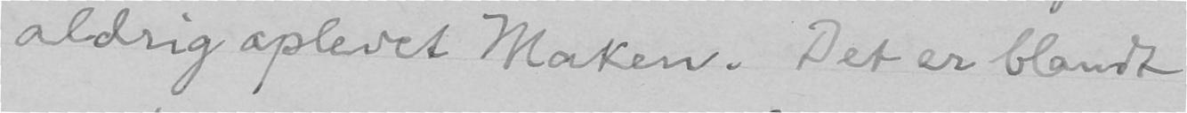aldrig oplevet Maken. Det er blandt
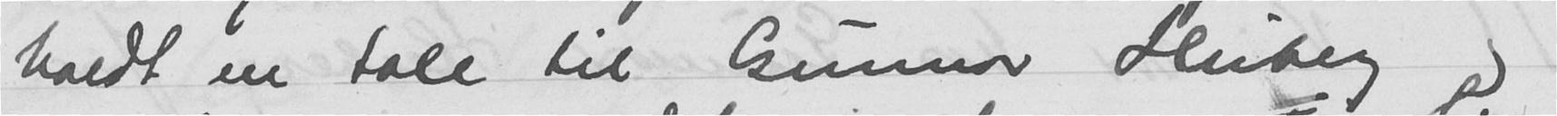holdt en tale til Gunnar Heiberg og
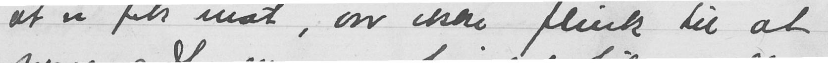at vi fik mat, var ikke flink til at
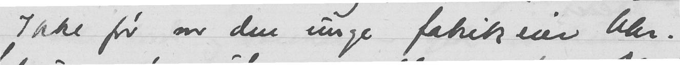Ikke før var den unge fabrikeier Chr.
